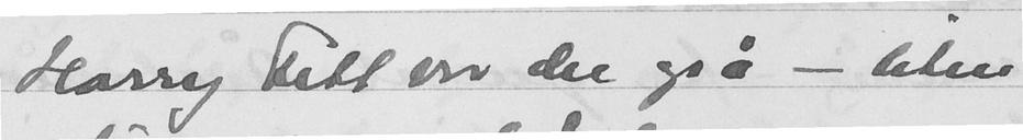Harry Fett var der også - liten
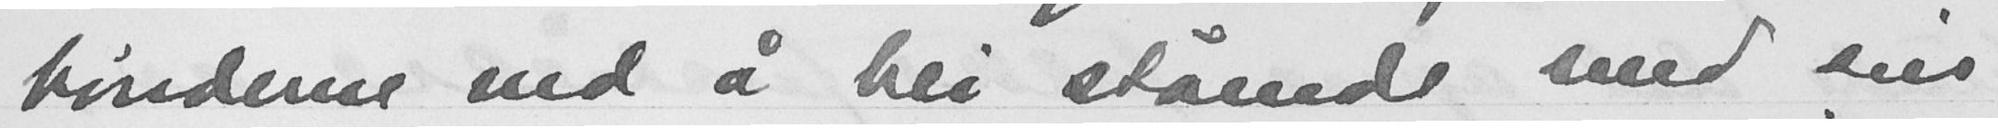bønderne ved å bli stående med sin
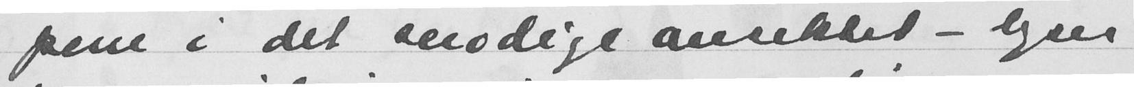pene i det snodige ansiktet - lyser
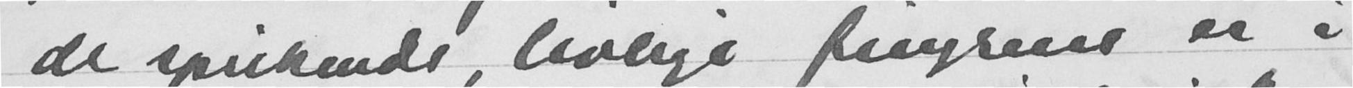de sprikende, livlige fingrene er i
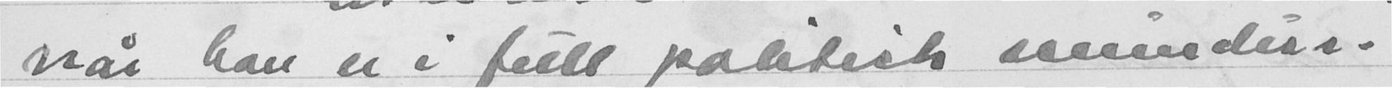når han er i fullt politisk seunders.
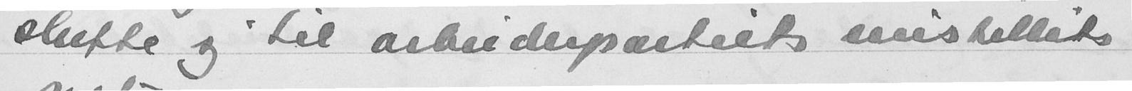slutte sig til arbeiderpartiets mistillits
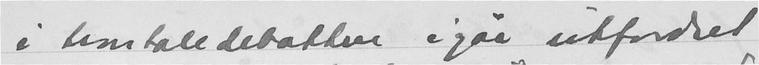i trostaledebatten igår utfordret
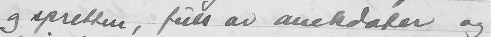og spretten, full av anekdoter og
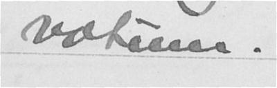votum.
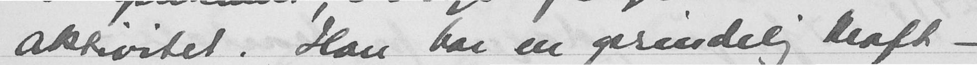aktivitet. Han har en oprindelig kraft -
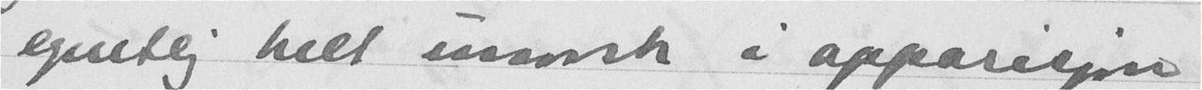egentlig helt unorsk i apparisjon
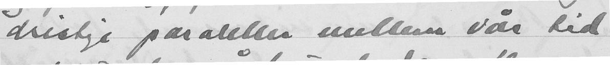dristige paraleller mellem vår tid
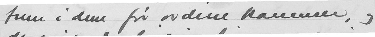frem i dem før ordene kommer, og
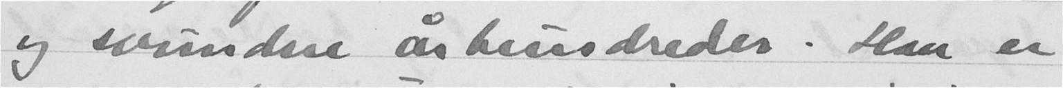og svundne århundrer. Han er
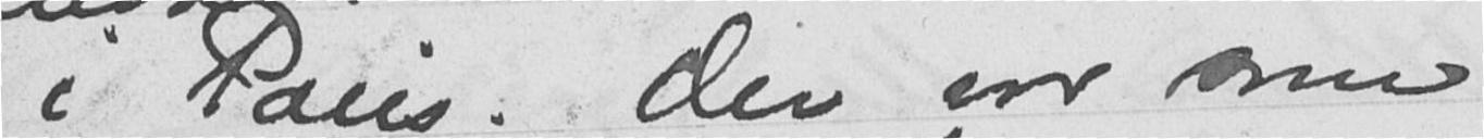i Paris. Der var som
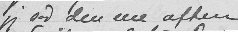jeg sad den ene aften
